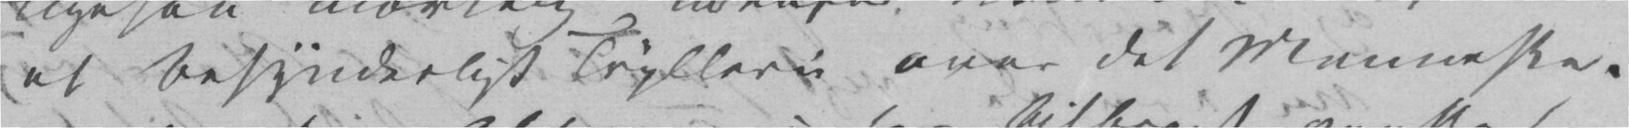et besynderligt Tryllerie over det Menneske.
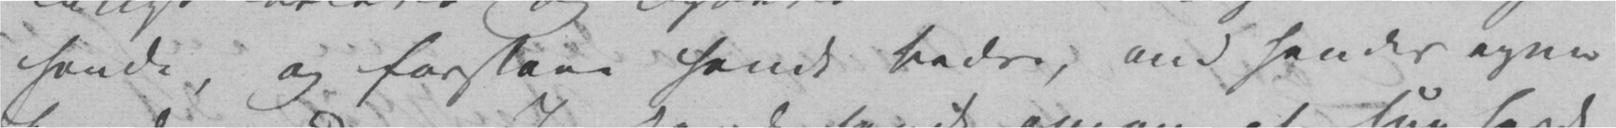hende, og forstaae hende bedre, end hendes egne
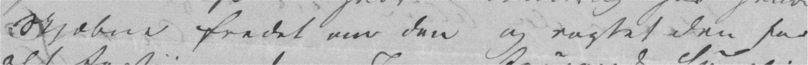Skjæbne fredet om den og vogtet den for
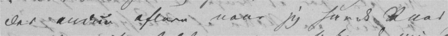der endnu oftere naar jeg havde Raad
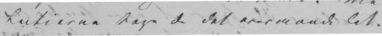Lectierne tage de det overmaade let.
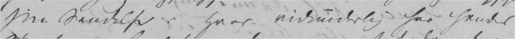sin Sendelse. Hvor vidunderlig har hendes
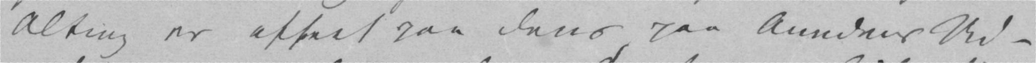Alting er offret paa dens paa Aandens Ud-
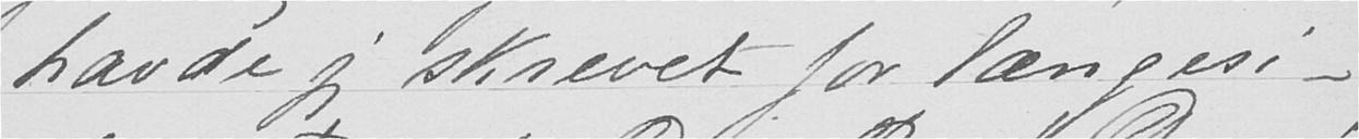havde jeg skrevet for længesi-
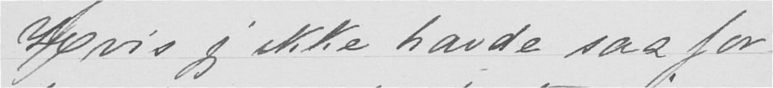Hvis jeg ikke havde saa for-
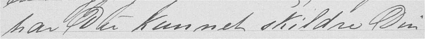har Du kunnet skildre Din
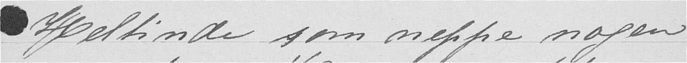Heltinde som neppe nogen
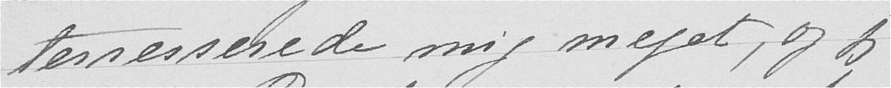terresserede mig meget, og jeg
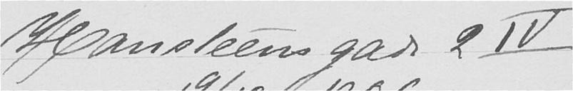Hansteens gade 2 IV
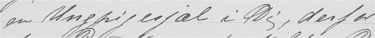en Ungpigesjæl i Dig, derfor
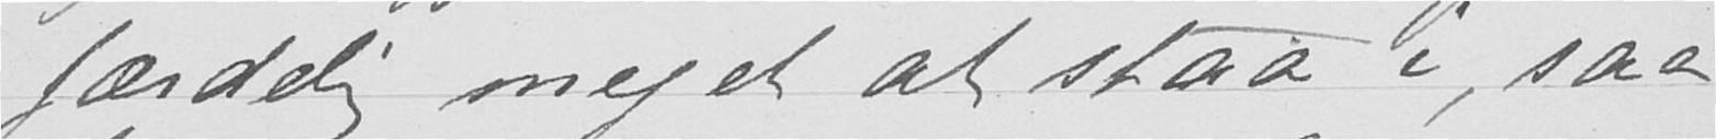færdelig meget at staa i, saa
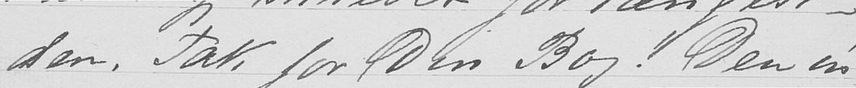den. Tak for Din Bog! Den in-
